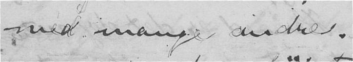med mange andre.
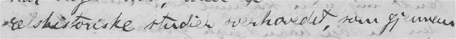retshistoriske studier overhovedet, som gjennem
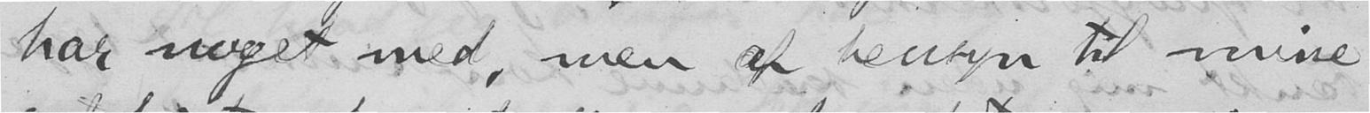har noget med, men af hensyn til mine
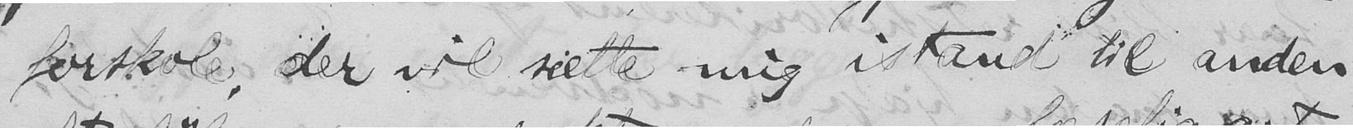forskole, der vil sætte mig istand til anden
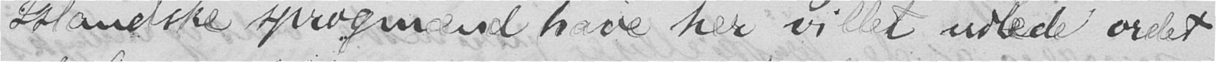Islandske sprogmænd have her villet udlede ordet
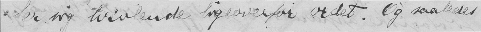der sig tvivlende ligeoverfor ordet. Og saaledes
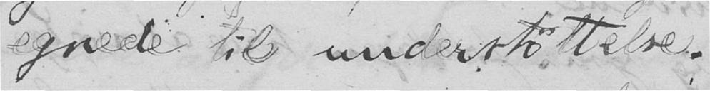egnede til understøttelse.
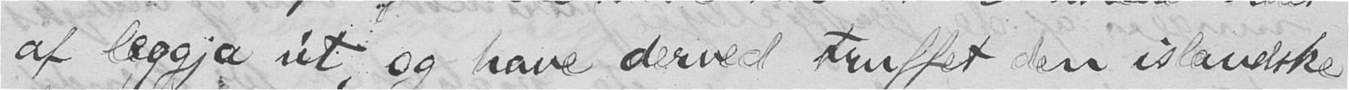af leggja út, og have derved truffet den islandske
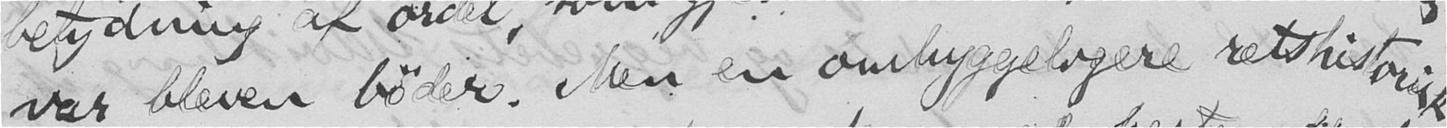var bleven bøder. Men en omhyggeligere retshistorisk
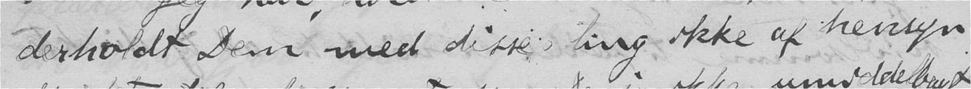derholdt Dem med disse ting ikke af hensyn
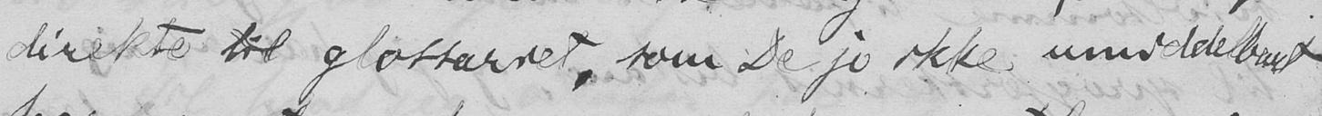direkte til glossariet, som De jo ikke umiddelbart
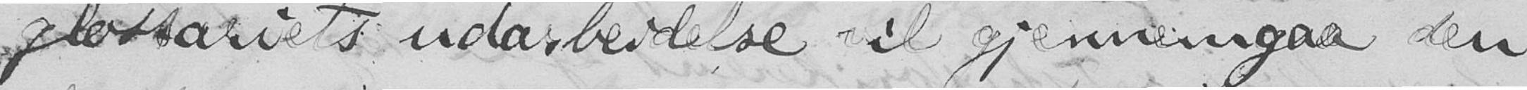glossariets udarbeidelse vil gjennemgaa den
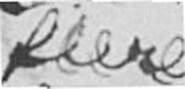flere
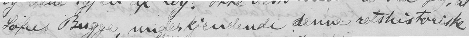Sofus Bugge, underkjendende denne retshistoriske
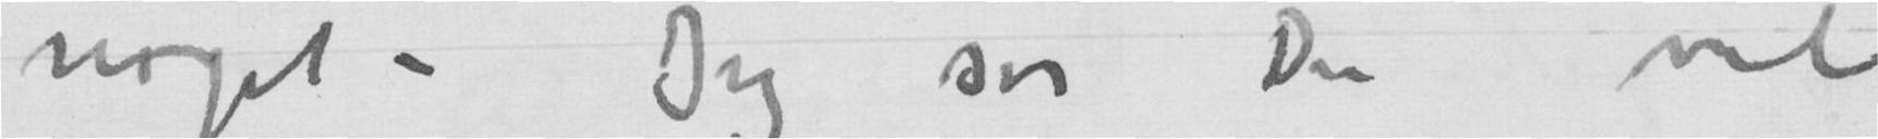noget – Jeg ser Du vil
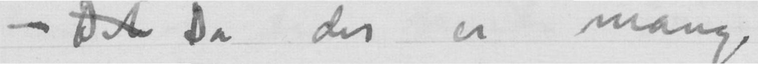–	Det Da der er mange
